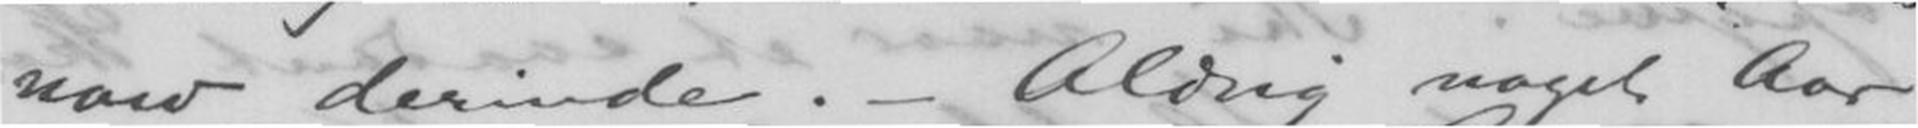now derinde. - Aldrig noget Aar
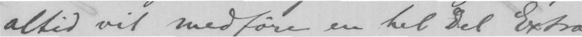altid vil medføre en hel Del Extraud-
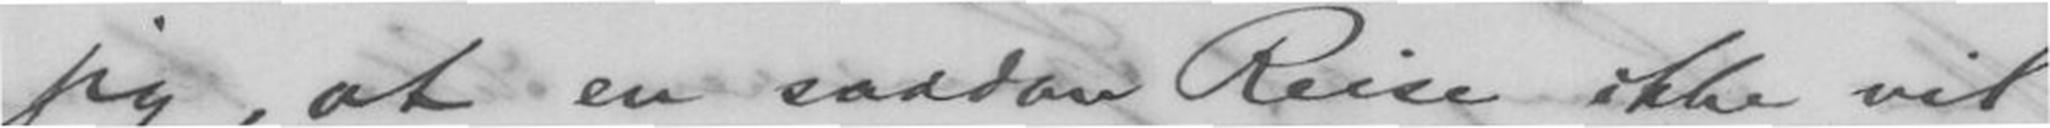jeg, at en saadan Reise ikke vil
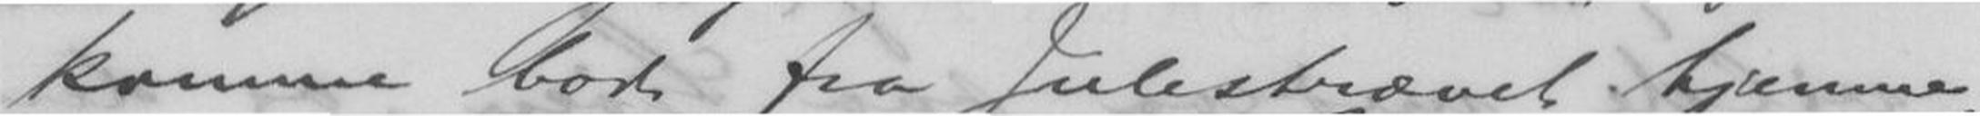komme bort fra Julestrævet hjemme,
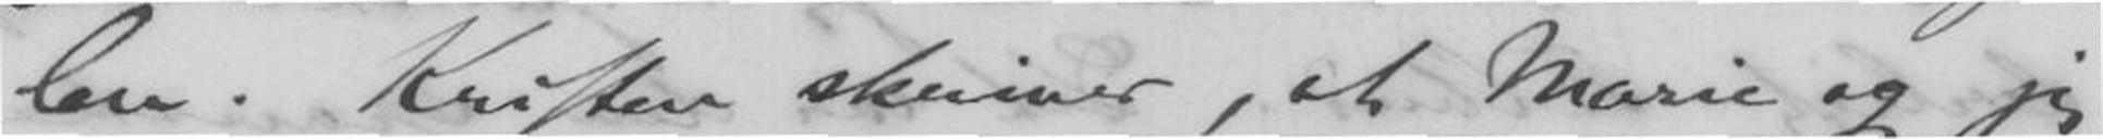len. Kristen skriver, at Marie og jeg
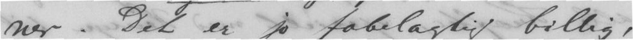ner. Det er jo fabelagtig billig,
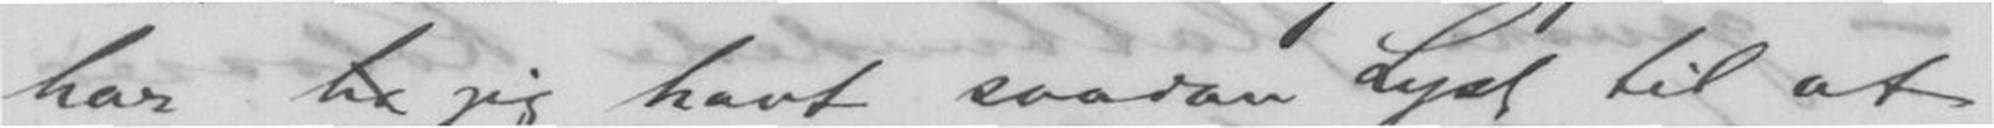har ha jeg havt saadan Lyst til at
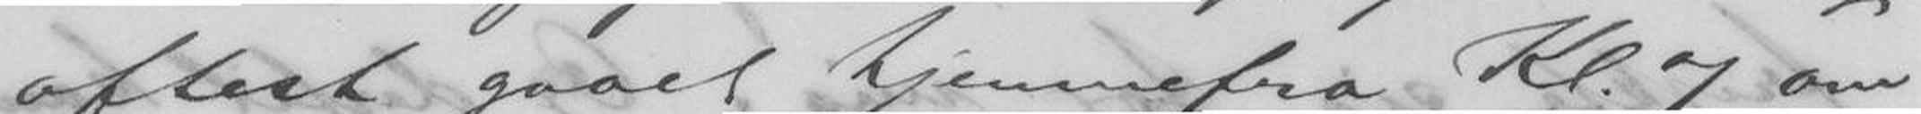oftest gaaet hjemmefra Kl. 7 om
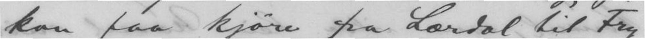kan faa kjøre fra Lærdal til Fry-
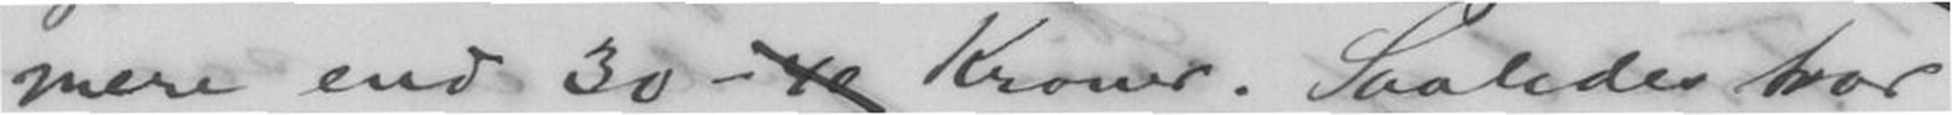mere end 30 - 40 Kroner. Saaledes tror
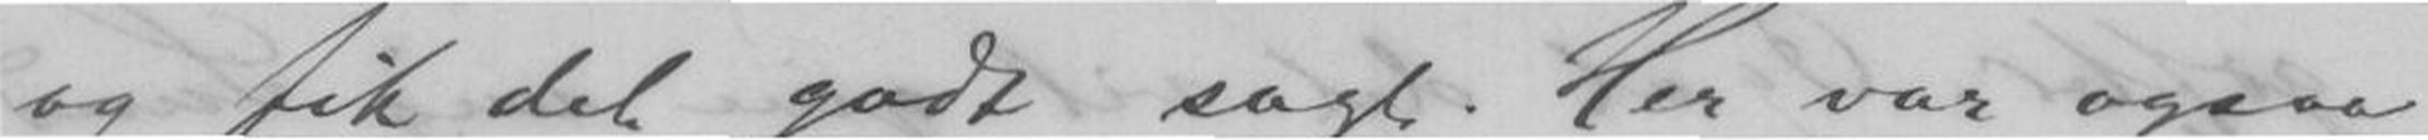og fik det godt sagt. Her var ogsaa
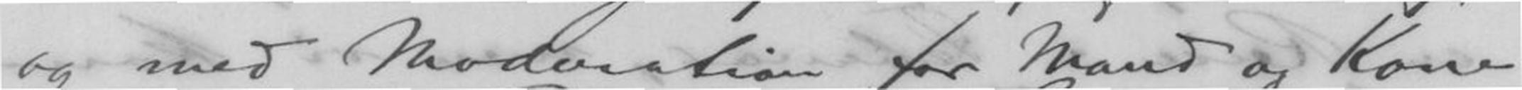og med Moderation for Mand og Kone
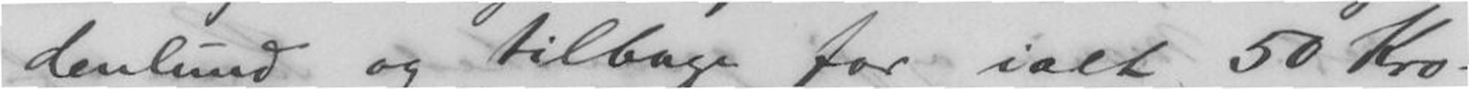denlund og tilbage for ialt 50 Kro-
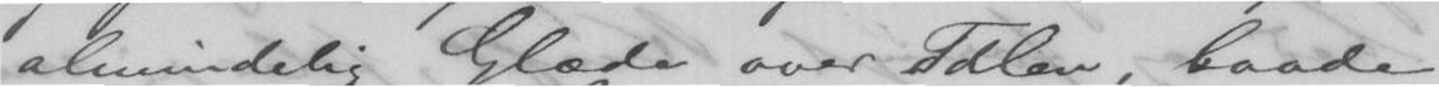almindelig Glæde over Talen, baade
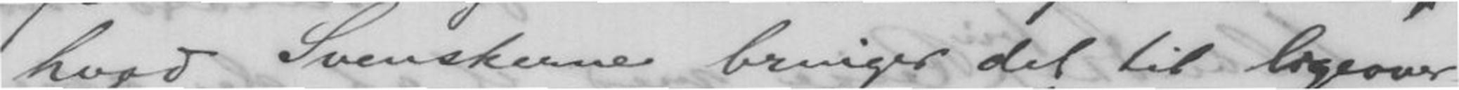hvad Svenskerne bringer det til ligeover-
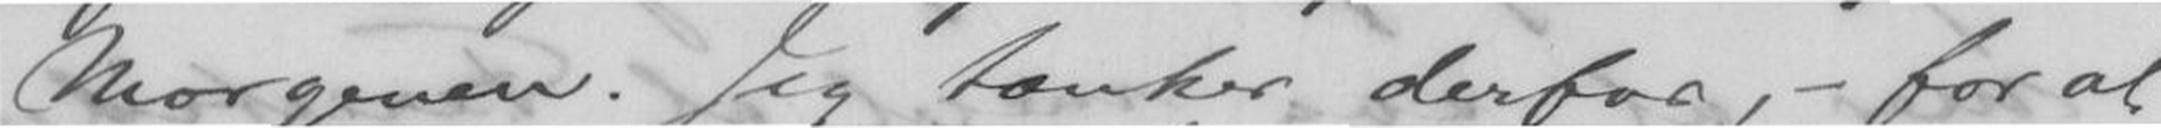Morgenen. Jeg tænker derfor, - for at
